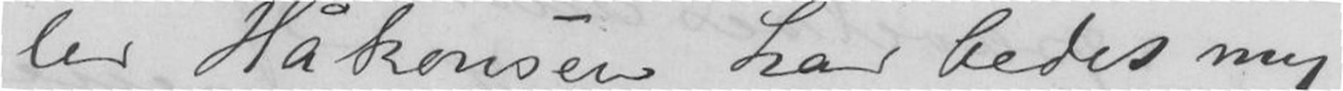ler Håkonsen har bedet mig
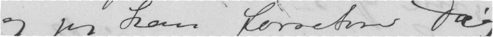og jeg kan forresten Dig
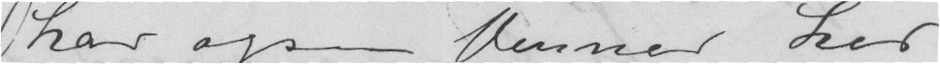har ogsaa Venner her
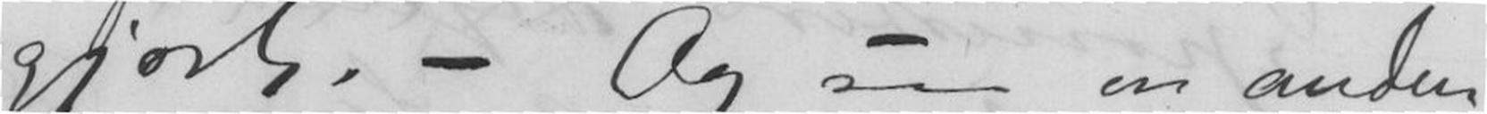gjort. - Og saa en anden
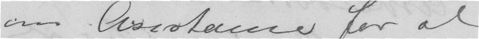om Asistanse for at
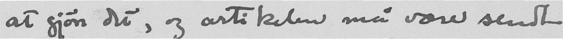at gjøre det, og artikelen må være sendt
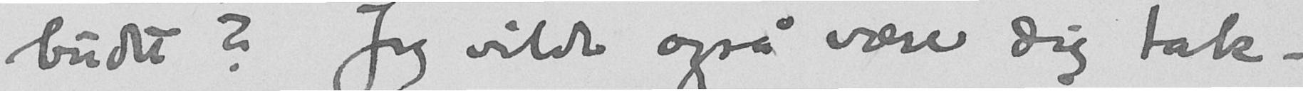budet? Jeg vilde også være dig tak-
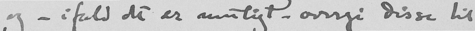og - ifald det er muligt - overgi disse til
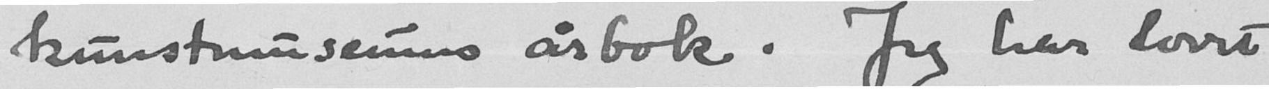kunstmuseums årbok. Jeg har lovet
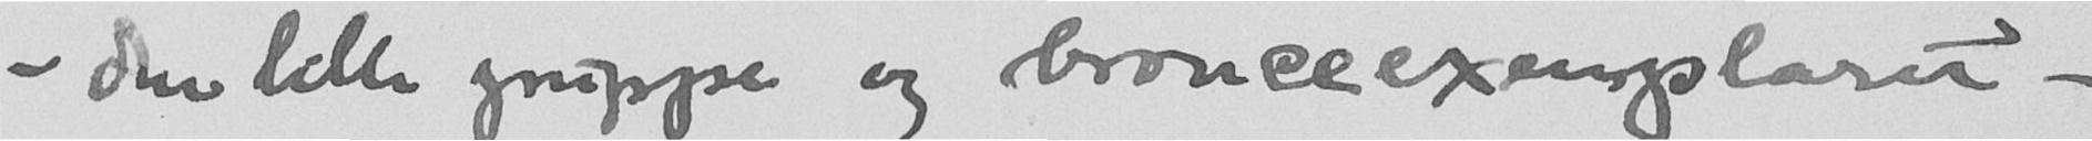- den lille gruppe og bronceexemplaret -
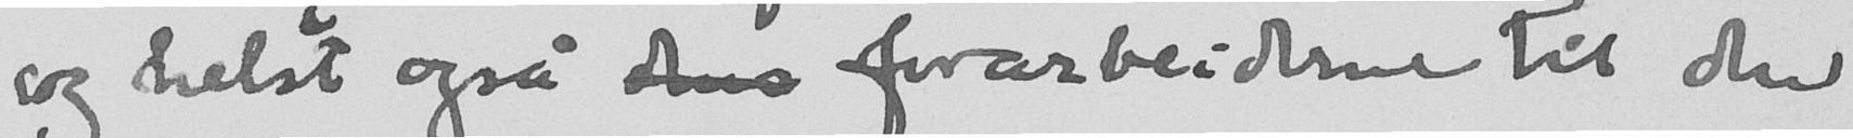og helst også dens forarbeiderne til den
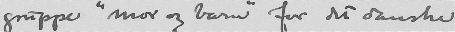gruppe "mor og barn" for det danske
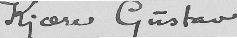Kjære Gustav
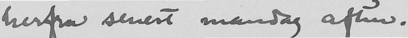herfra senest mandag aften.
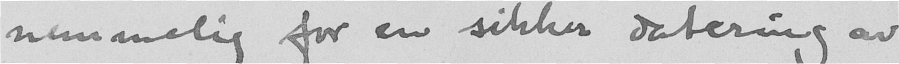nemmelig for en sikker datering av
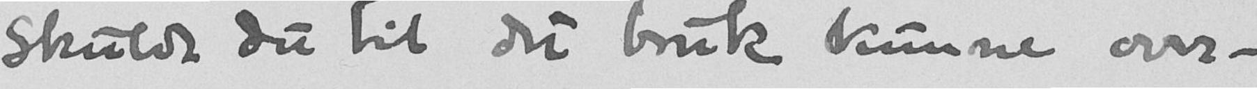Skulde du til det bruk kunne over-
